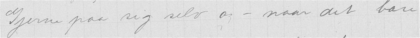Gjerne paa sig selv og - naar det bare
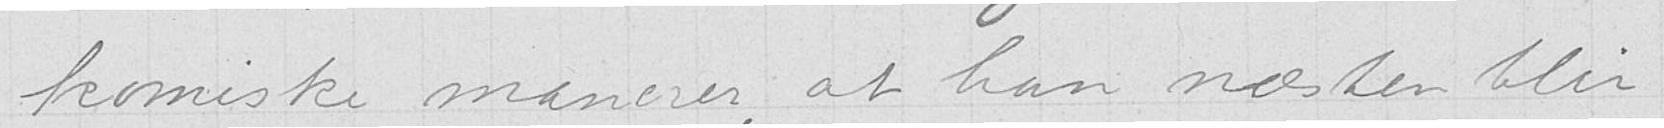komiske manerer, at han næsten blir
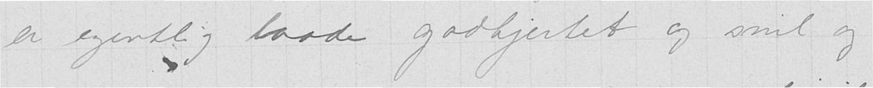er egentlig baade godhjertet og snil og
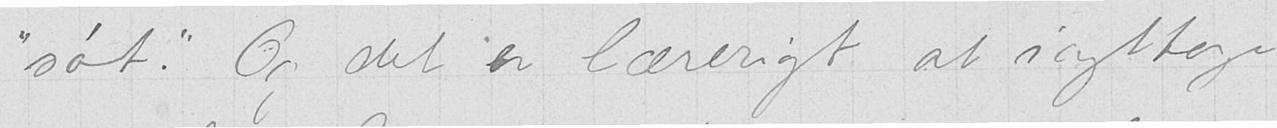"søt". Og det er lærerigt at iagttage
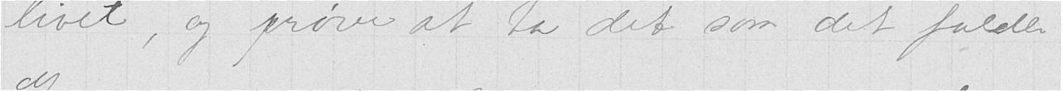livet, og prøve at ta det som det falder.
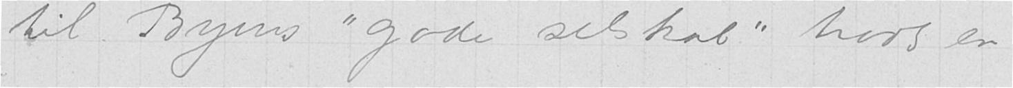til Byens "gode selskab" trods en
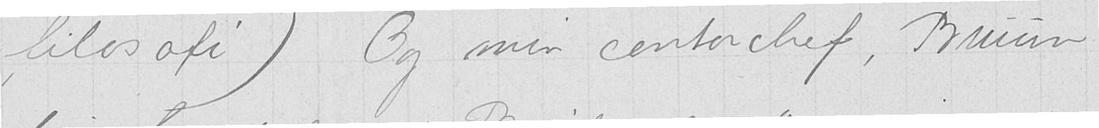filosofi). Og min kontorchef, Bruun
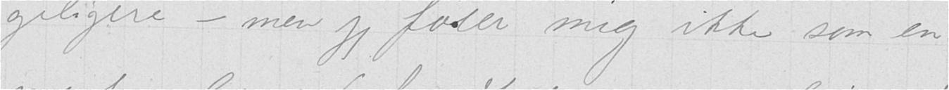geligere - men jeg føler mig ikke som en
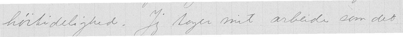høitidelighed. Jeg tager mit arbeide som det
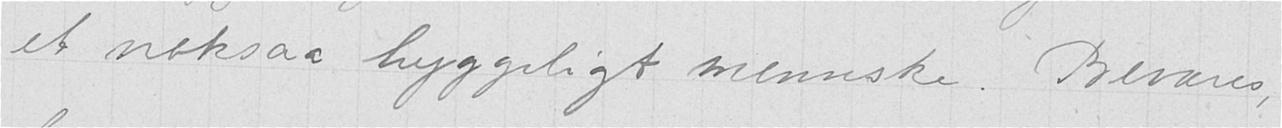et noksaa hyggeligt menneske. Bevares,
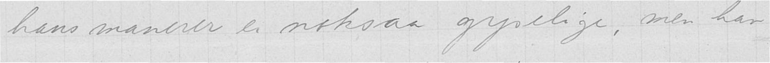hans manerer er noksaa gryselige, men han
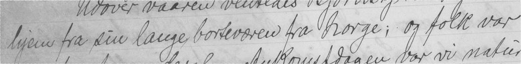hjem fra sin lange borteværen fra Norge; og folk var
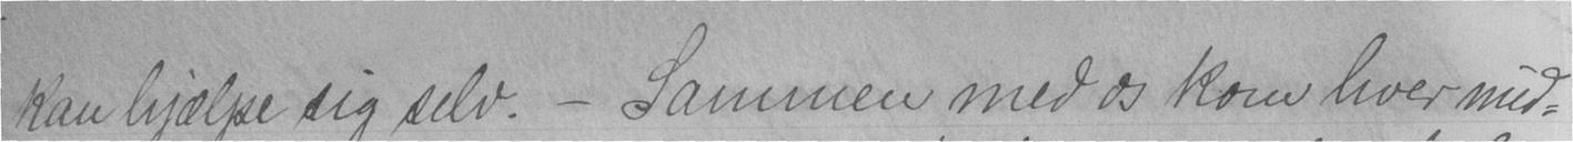kan hjælpe sig selv. - Sammen med os kom hver mid-
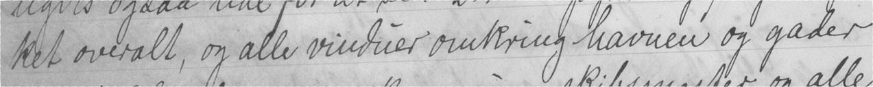ket overalt, og alle vinduer omkring havnen og gader
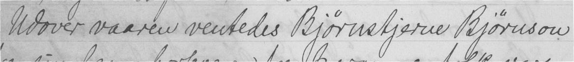Udover vaaren ventedes Bjørnstjerne Bjørnson
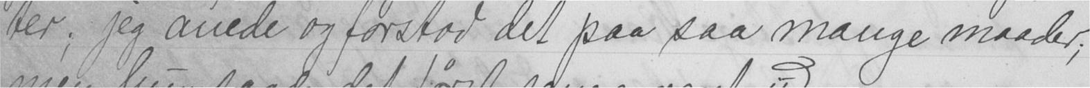ter; jeg anede og forstod det paa saa mange maader;
